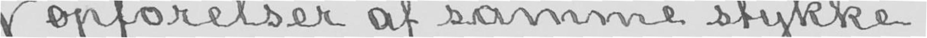opførelser af samme stykke.
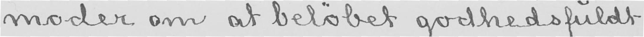moder om at beløbet godhedsfuldt
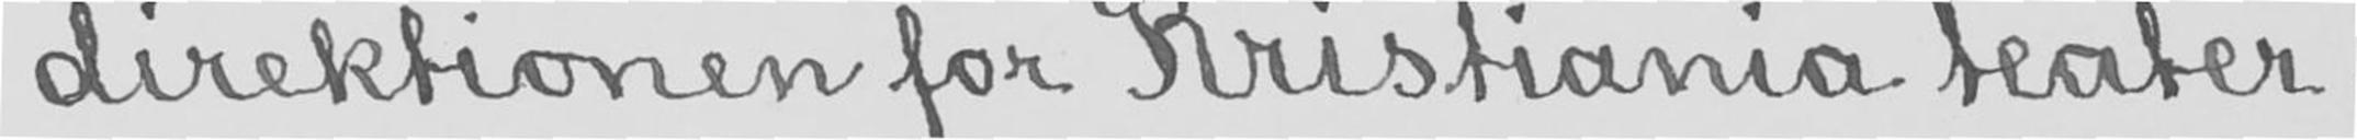direktionen for Kristiania teater.
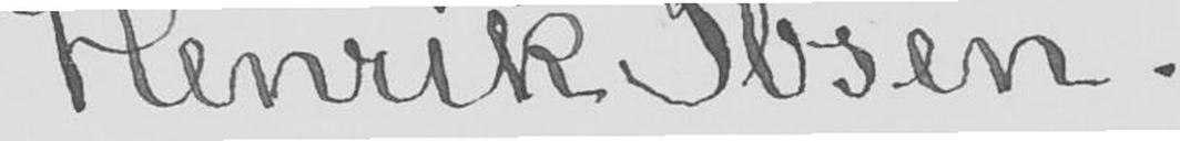Henrik Ibsen.
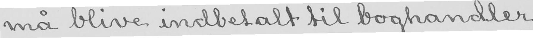må blive indbetalt til boghandler
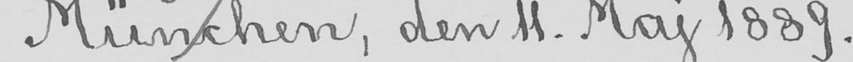München, den 11. Maj 1889.
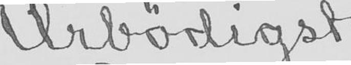Ærbødigst
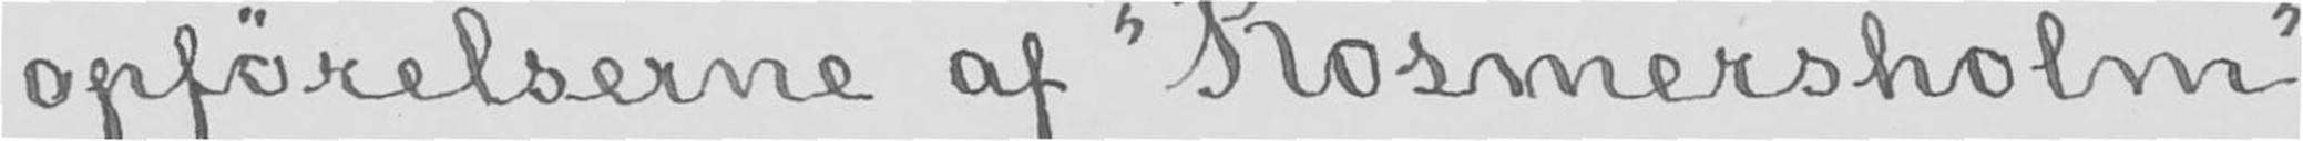opførelserne af «Rosmersholm»
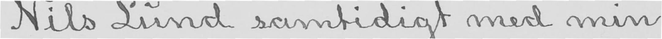Nils Lund samtidigt med min
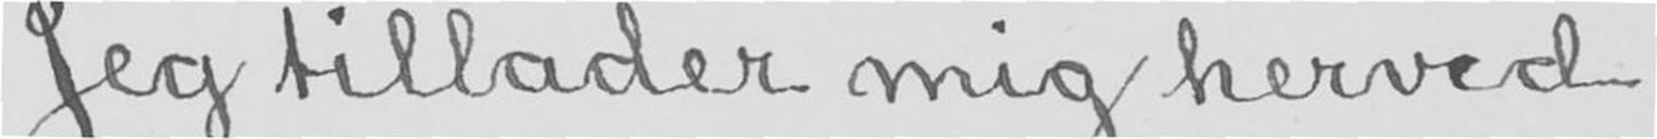Jeg tillader mig herved
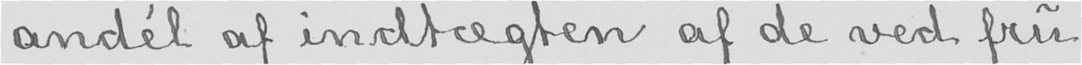andél af indtægten af de ved fru
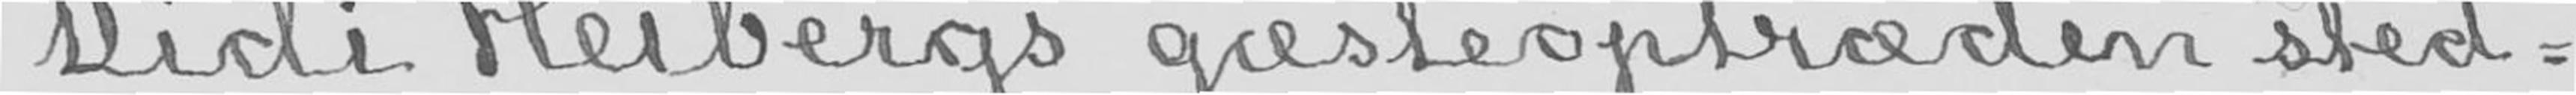Didi Heibergs gæsteoptræden sted-
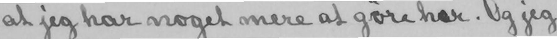at jeg har noget mere at gøre her. Og jeg
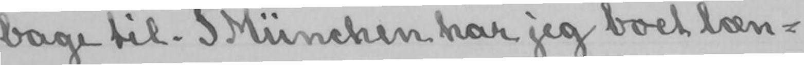bage til. I München har jeg boet læn-
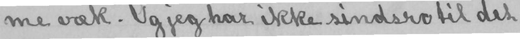me væk. Og jeg har ikke sindsro til det
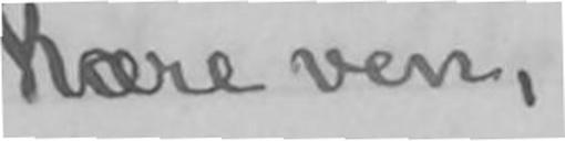Kære ven,
